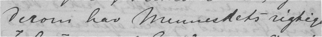derom har Menneskets rigtige
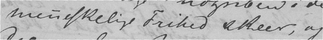menneskelige Frihed skeer, og
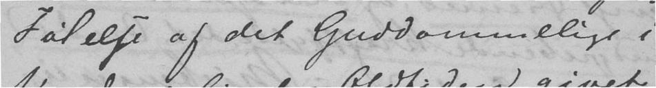Følelse af det Guddommelige i
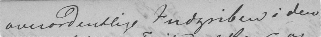overordentlige Indgriben i den
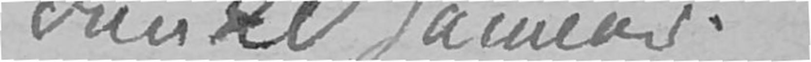Den 20 Januar.
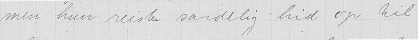men hun reiste sandelig hid op til
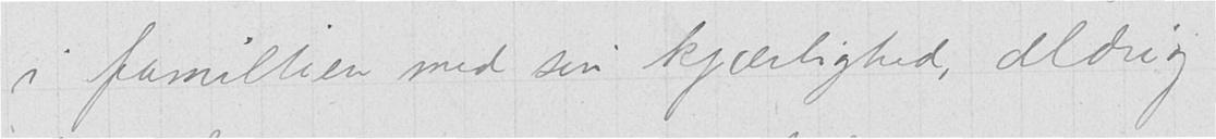i familien med sin kjærlighed, aldrig
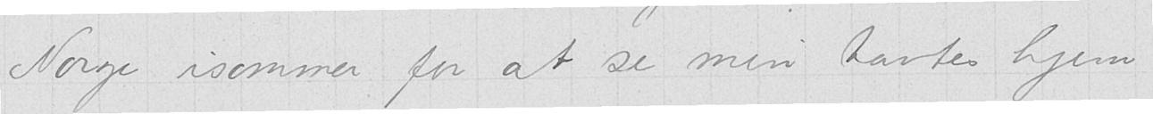Norge isommer for at se min tantes hjem
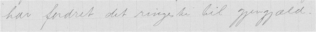har fordret det ringeste til gjengjæld.
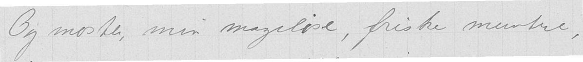Og moster, min mageløse, friske muntre,
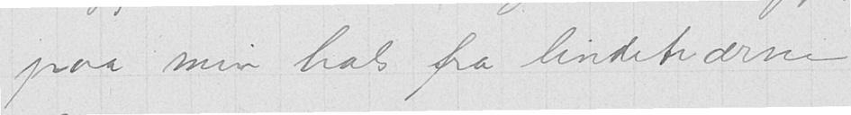paa min hals fra lindetærne.
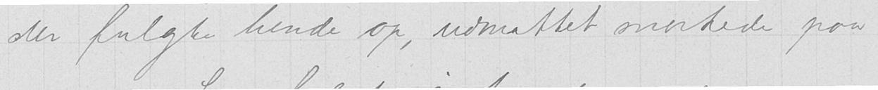der fulgte hende op, udmattet snorkede paa
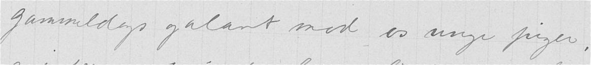gammeldags galant mod os unge piger,
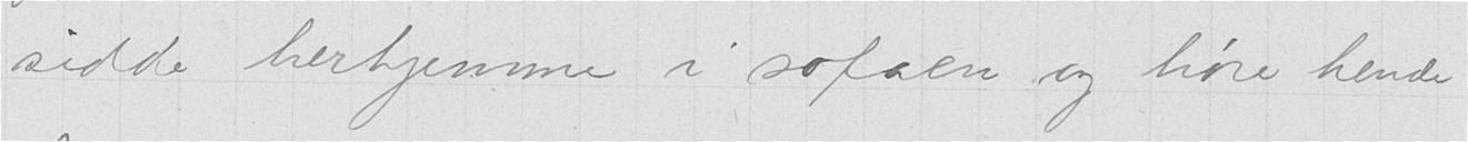sidde herhjemme i sofaen og høre hende
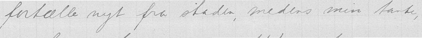fortælle nyt fra staden, medens min tante,
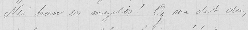Nei han er mageløs! Og saa det du,
